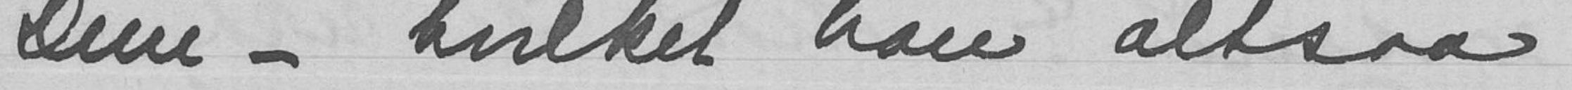Dem - hvilket han altsaa
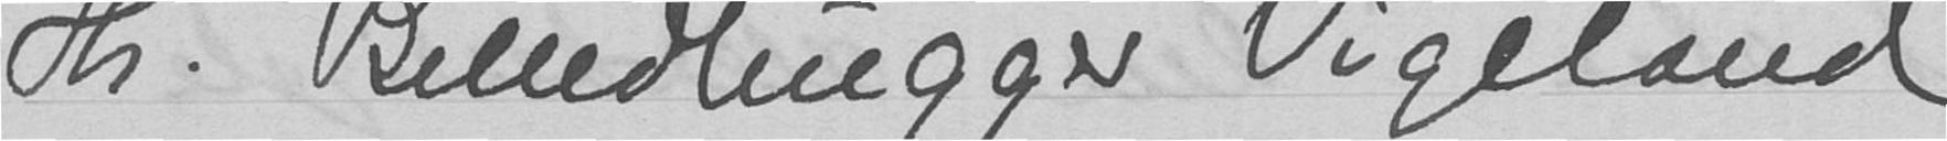Hr. Billedhugger Vigeland
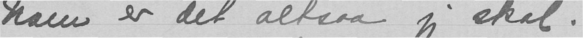ham er det altsaa jeg skal.
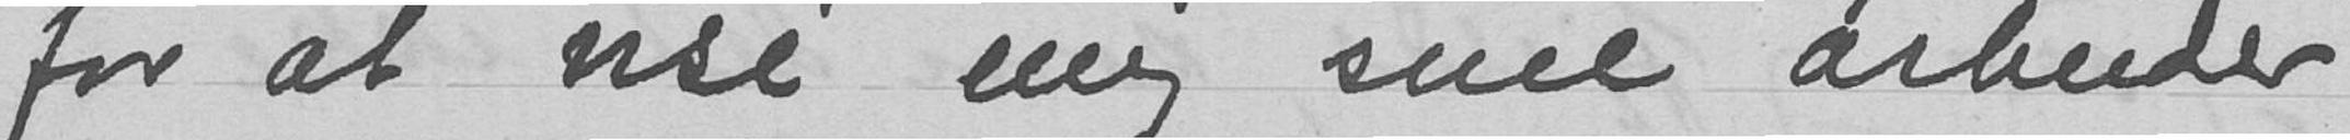for at vise mig sine arbeider
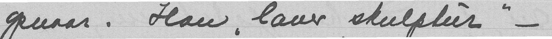opnaar. Han "laver skulptur" -
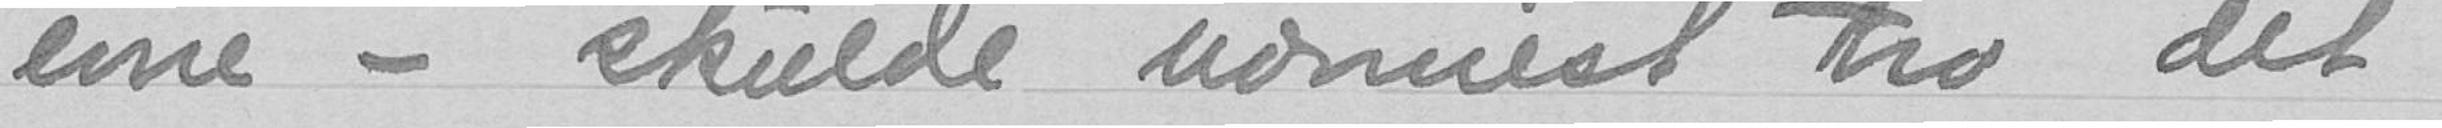evne - skulde nærmest tro det
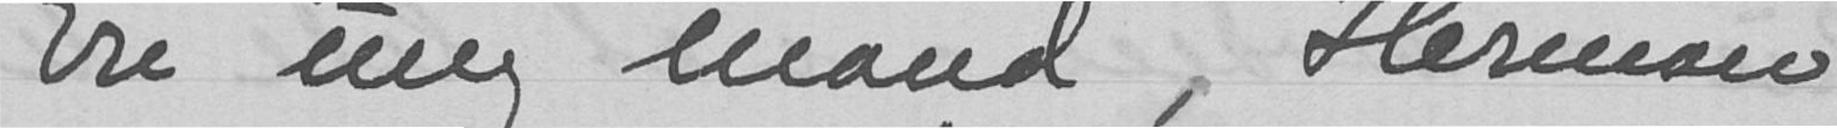En ung mand, Herman
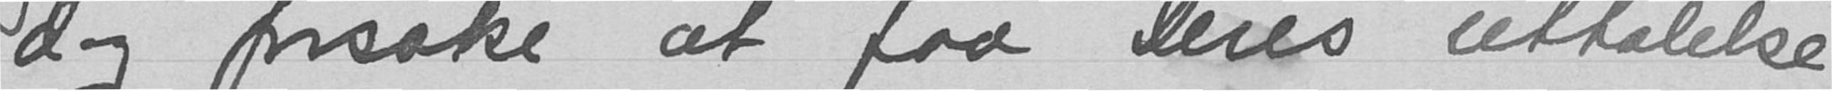dag forsøke at faa Deres uttalelse
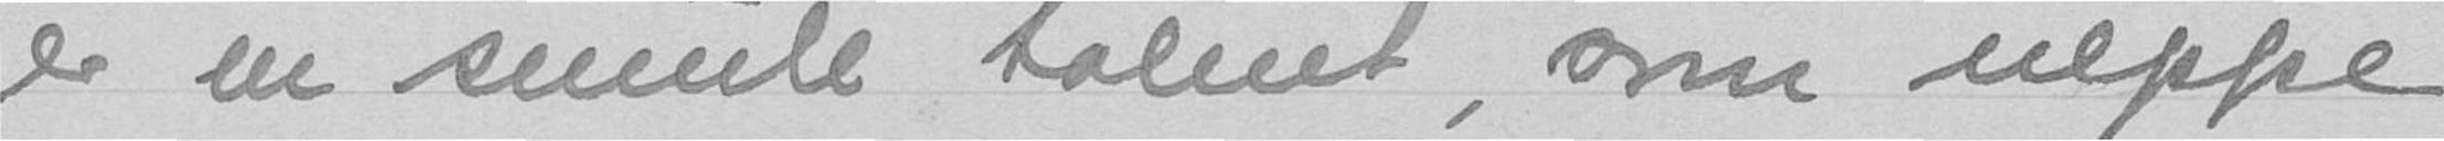er en smule talent, som neppe
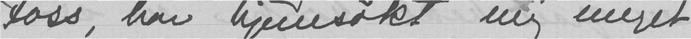Foss, har hjemsøkt mig meget
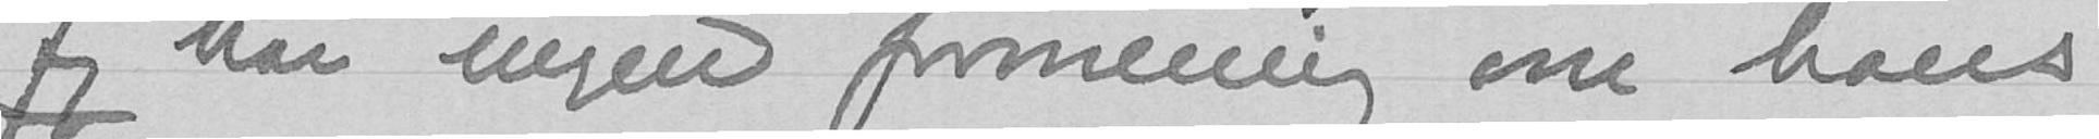Jeg har ingen formening om hans
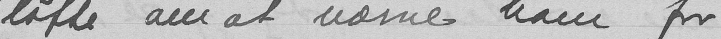løfte om at nævne ham for
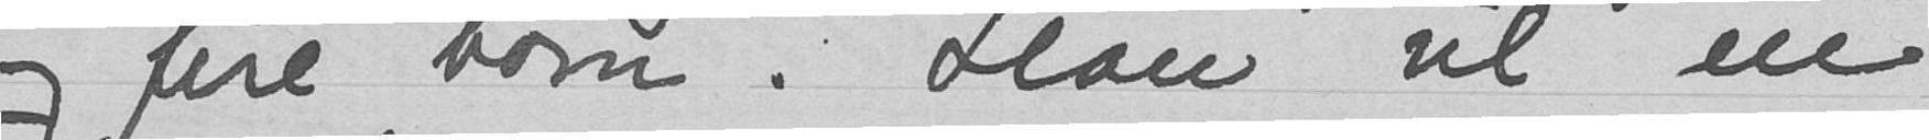og fire børn. Han vil en
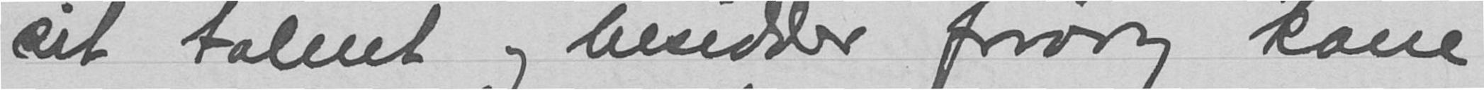sit talent og besidder forøvrig kone
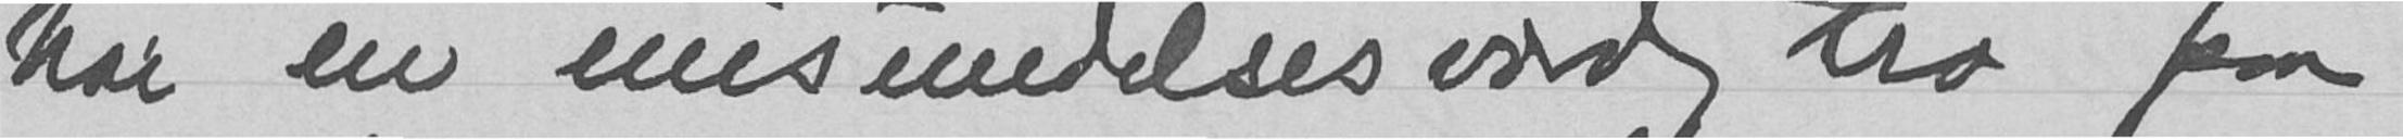har en misundelsesverdig tro paa
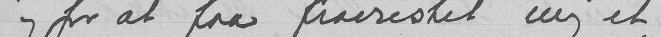og for at faa fravristet mig et
